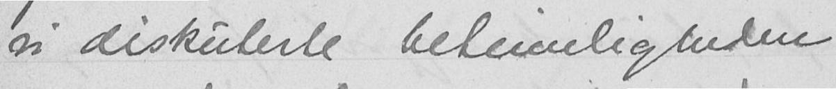vi diskuterte betimeligheden
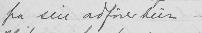fra sin ordførertur -
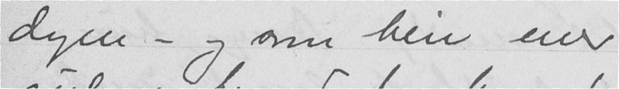dagen - og som blir mer
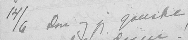14/6 Don og jeg ganske
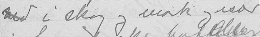ved i skog og mark og især
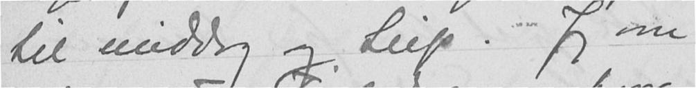til middag og Seip. Jeg om
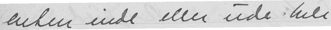enten inde eller ude hele
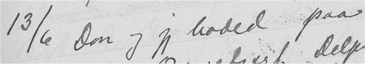13/6 Don og jeg baded paa
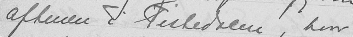aftenen i Tistedalen, hvor
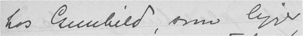hos Gunhild, som ligger
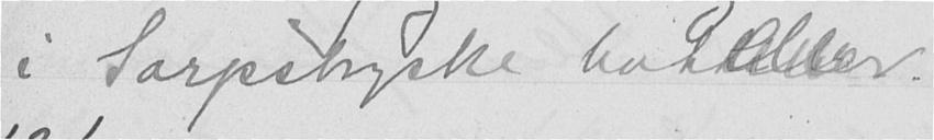i Sarpsborgske hoteller.
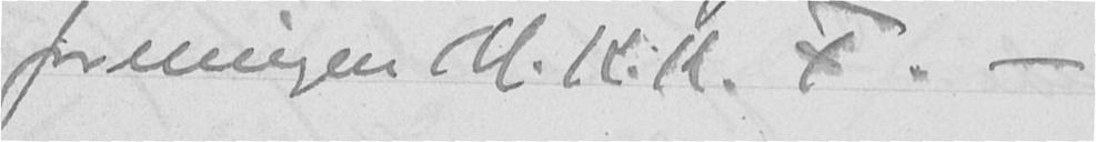foreningen M. K. K. F. -
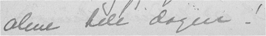alene hele dagen!
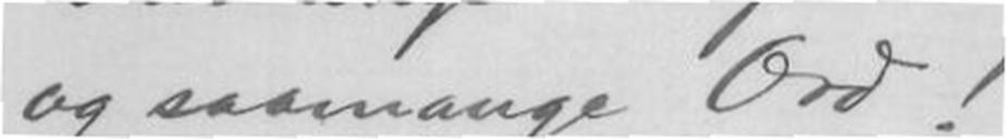og saamange Ord!
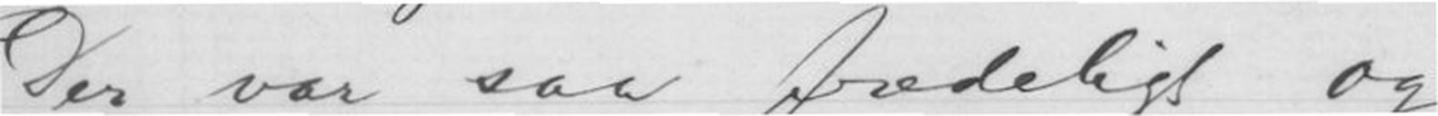Der var saa fredeligt og
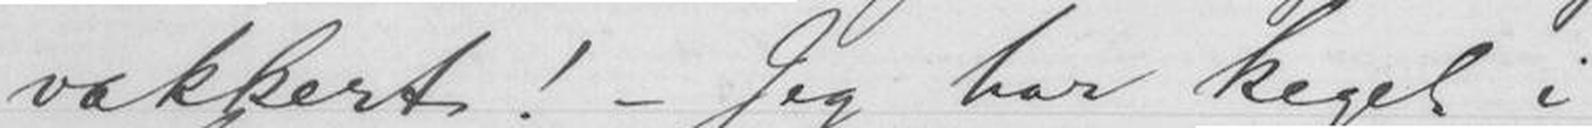vakkert! - Jeg har keget i
